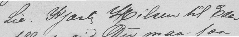Lie. Kjærlig Hilsen til Eder
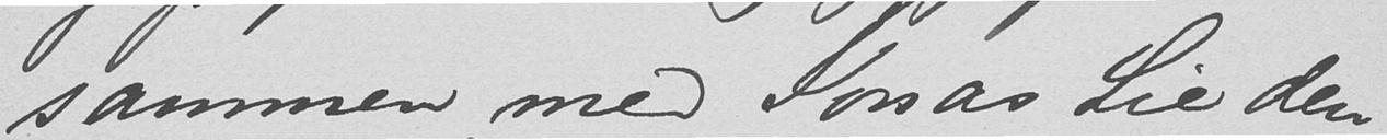sammen med Jonas Lie den
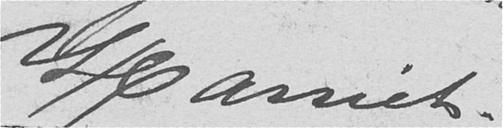Harriet.
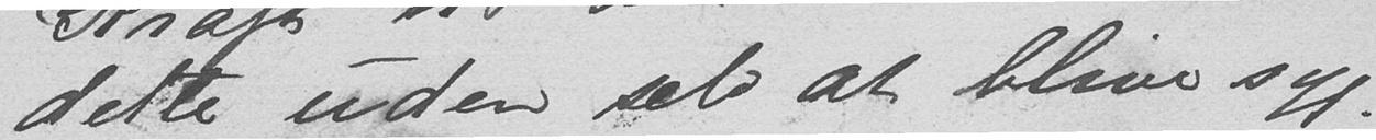dette uden selv at blive syg.
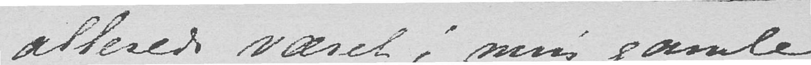allerede været i min gamle
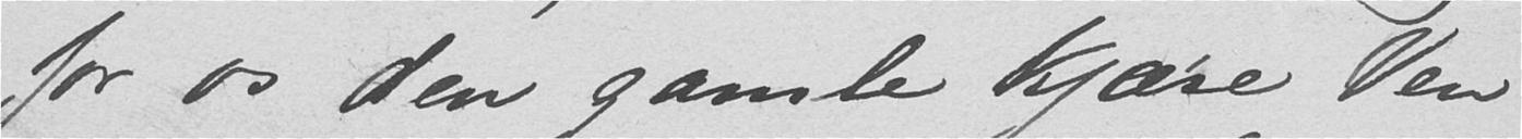for os den gamle Kjære Ven
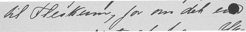til Fleskum, for om det end
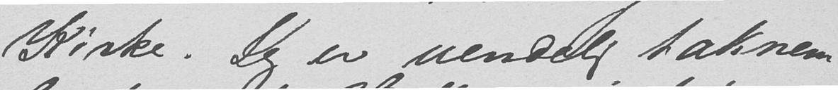Kirke. Jeg er uendelig taknem-
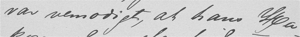var vemodigt, at hans Hu-
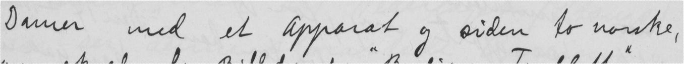Damer med et Apparat og siden to norske,
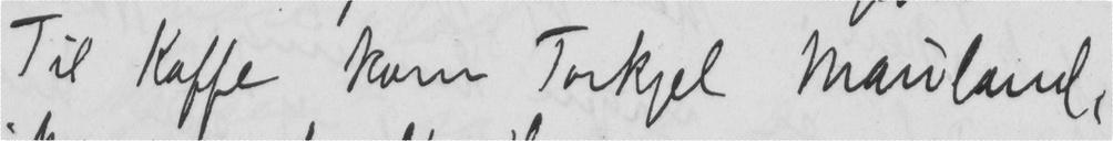Til Kaffe kom Torkgel Mauland,
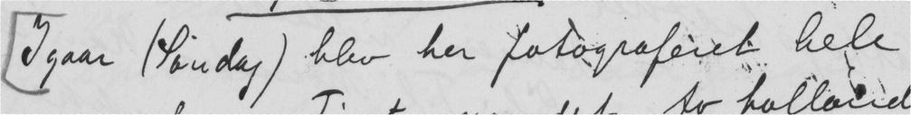Igaar (Søndag) blev her fotograferet hele
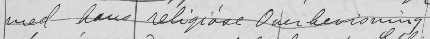med hans religiøse Overbevisning
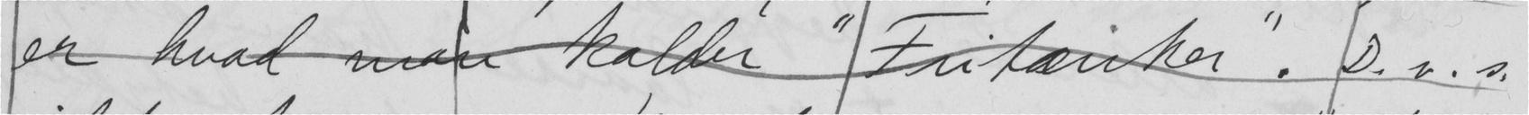er hvad man kalder "Fritænker". D.v.s.
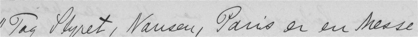"Tag Styret, Nansen, Paris er en Messe
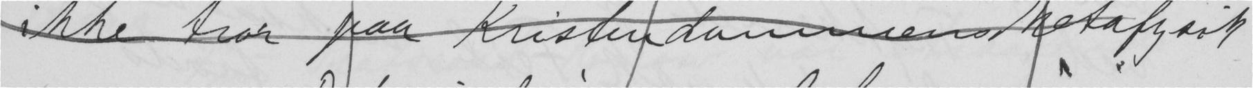ikke tror paa Kristindommens Metafysik
